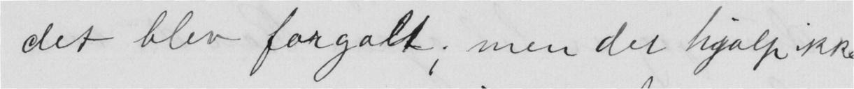det blev forgalt, men det hjælper ikke
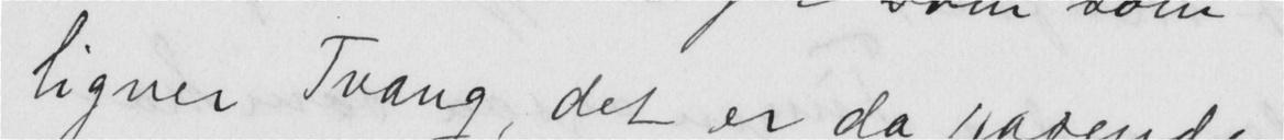ligner Tvang, det er da varende
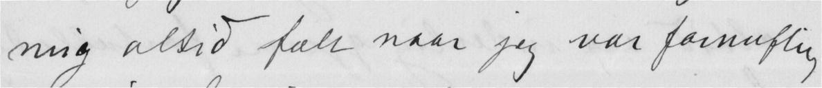mig altid fælt naar jeg var fornuflig
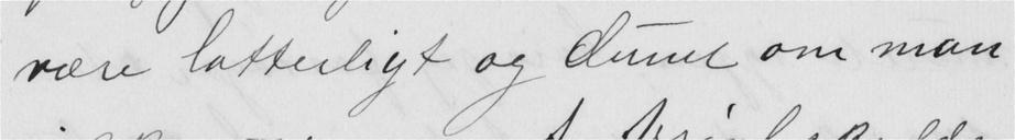være latterligt og dùmt om man
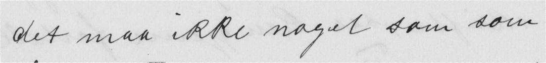det maa ikke noget som som
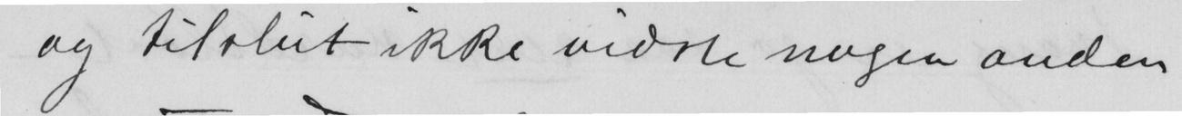og tilslut ikke vidste nogen anden
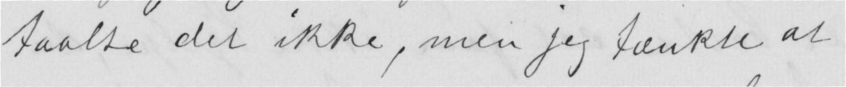taalte det ikke, men jeg tænkte at
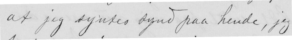at jeg syntes synd paa hende, jeg
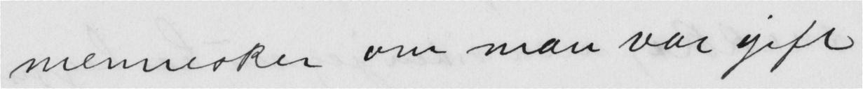mennesker om man var gift
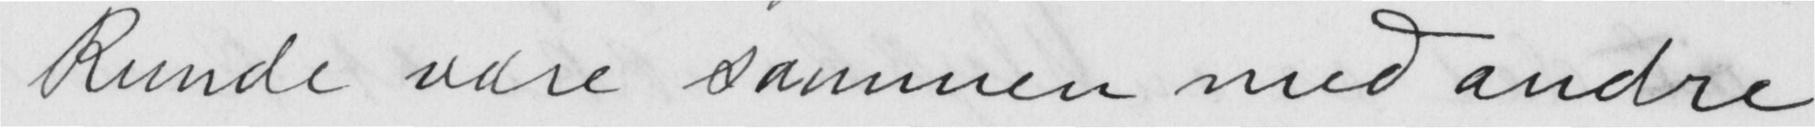Kunde være sammen med andre
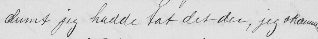dumt jeg hadde tat det der, jeg skammer
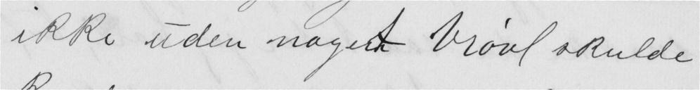ikke uden noget Vrøvl skulde
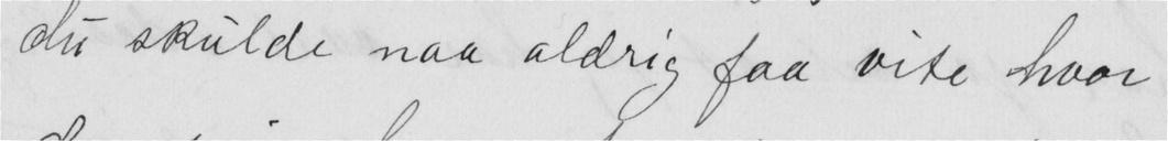du skulde naa aldrig faa vite hvor
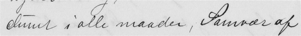dumt i alle maader, Samvær af
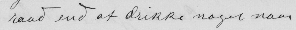raad end at drikke noget naar
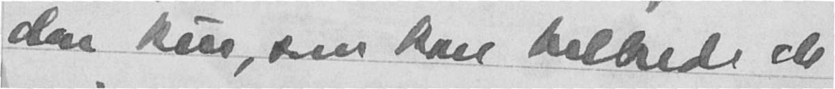den kur, som kan herlbrede de
